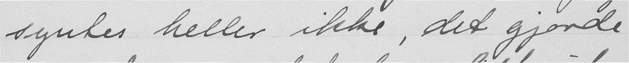syntes heller ikke, det gjorde
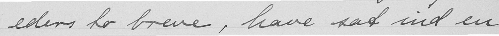eders to breve, have sat ind en
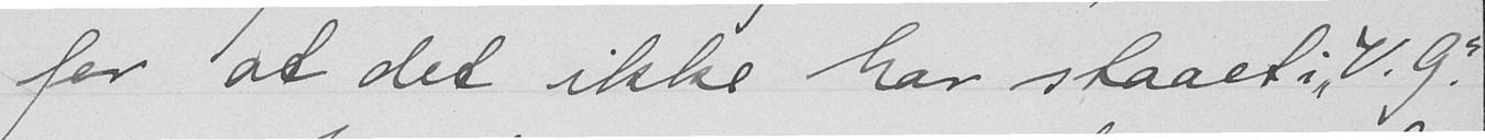for at det ikke har staaet i "V. G."
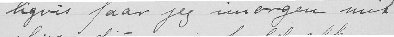ligvis faar jeg imorgen mit
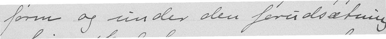form og under den forudsætning
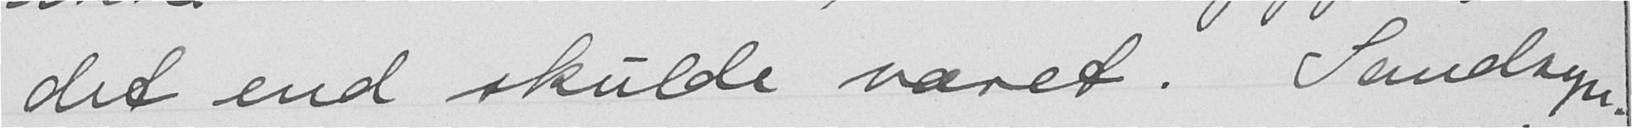det end skulde været. Sandsyn-
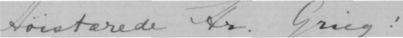Høistærede Hr. Grieg!
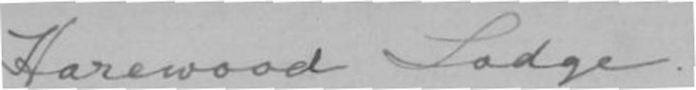Harewood Lodge.
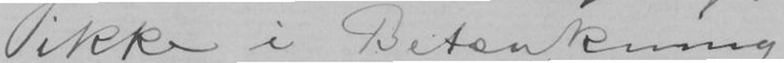ikke i Betenkning
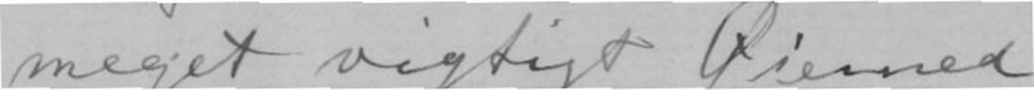meget vigtigt Øiemed.
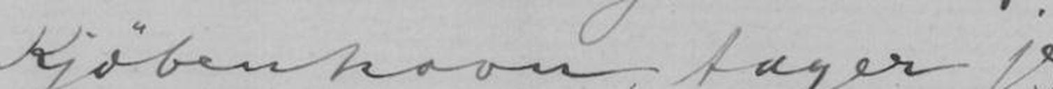Kjøbenhavn tager jeg
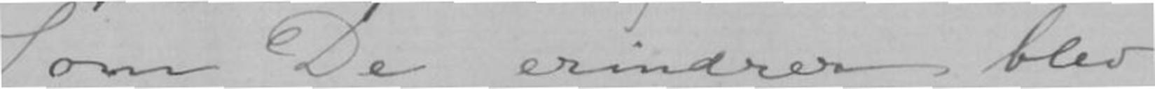Som De erindrer blev
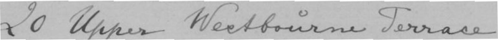20 Upper Westbourne Terrace
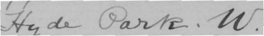Hyde Park. W.
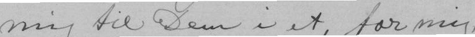mig til Dem i et, for mig,
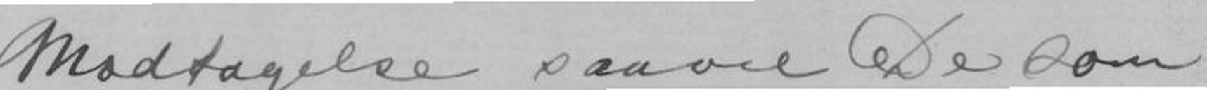Modtagelse saavel De som
